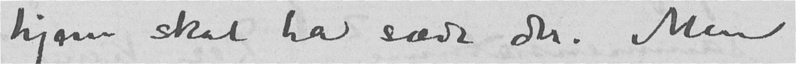hjem skal ha sæde der. Men
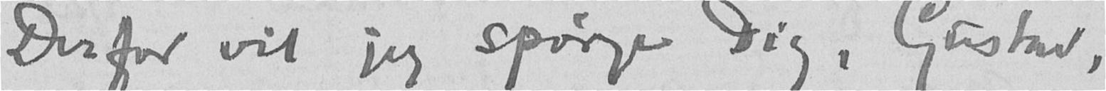Derfor vil jeg spørge Dig, Gustav,
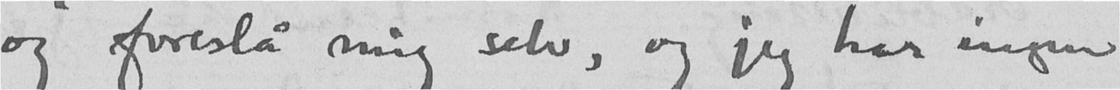og foreslå mig selv, og jeg har ingen
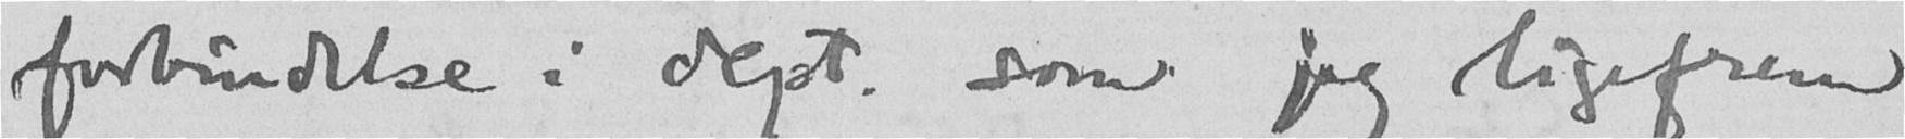forbindelse i dept. som jeg ligefrem
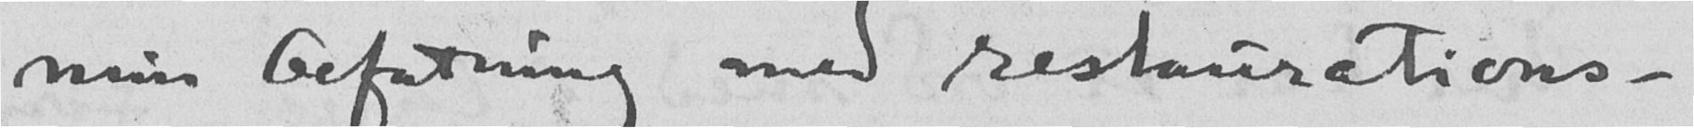min befatning med restaurations-
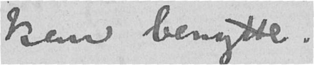kan benytte.
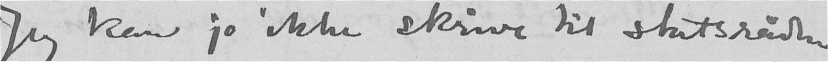Jeg kan jo ikke skrive til statsråden
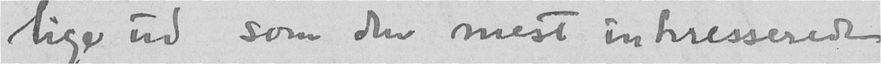lige ud som den mest interesserede
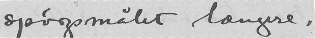spørgsmålet længere.
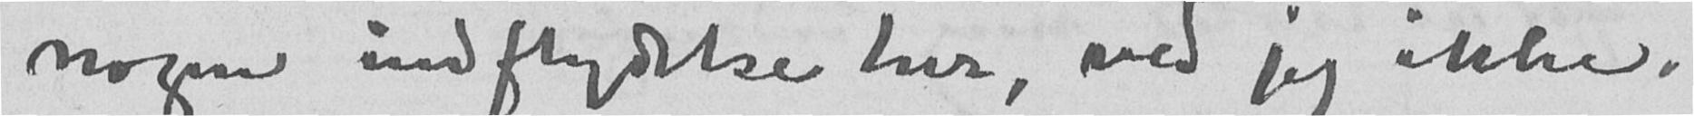nogen indflydelse her, ved jeg ikke.
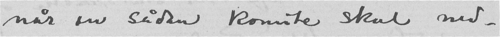når en sådan komite skal ned-
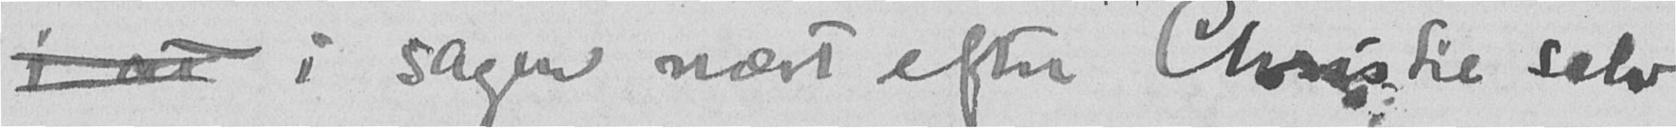i at i sagen nært efter Christie selv
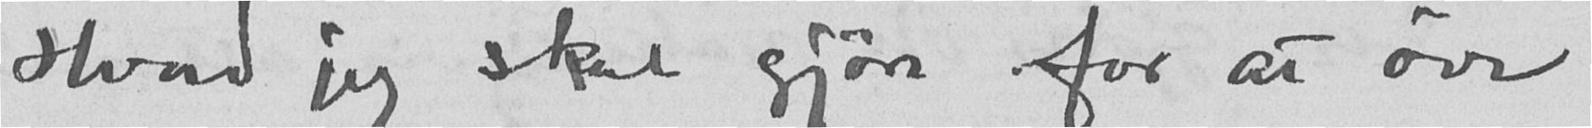Hvad jeg skal gjøre for at øve
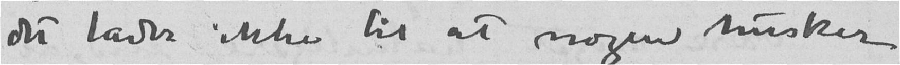det lader ikke til at nogen husker
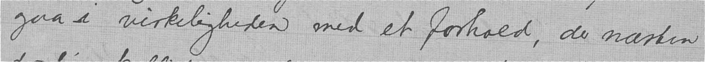gaa i virkeligheden med et forhold, de næsten
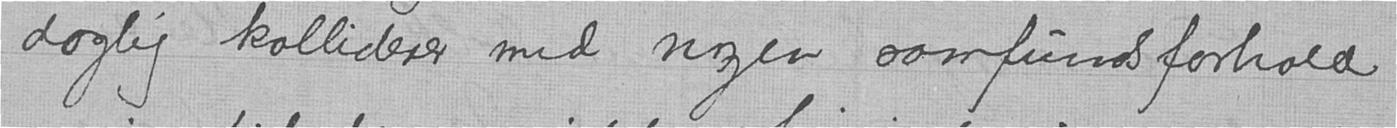daglig kolliderer med nogen samfundsforhold
